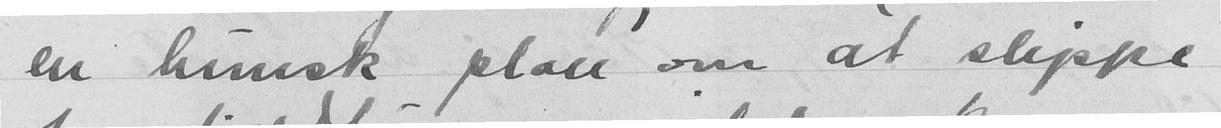en lumsk plan om at slippe
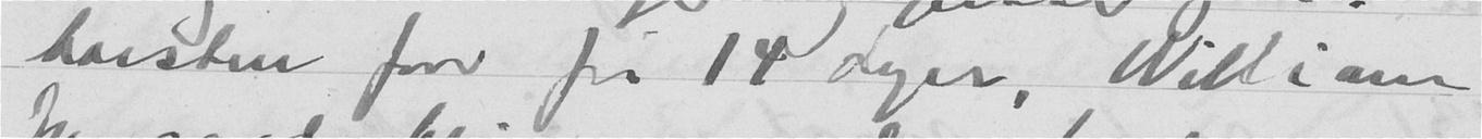Carsten faar for 14 dager, William
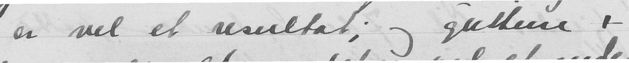er vel et resultat; og guttene -
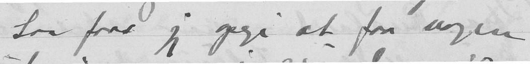Saa faar jeg opgi at faa nogen
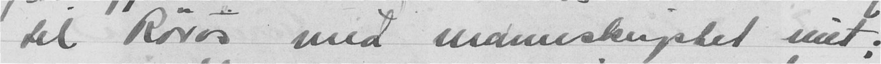til Røros med manuskriptet mit;
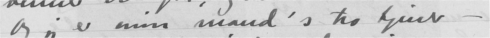Og jeg er min mand's tro tjener -
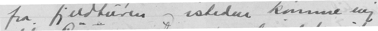fra fjeldturen og isteden komme mig
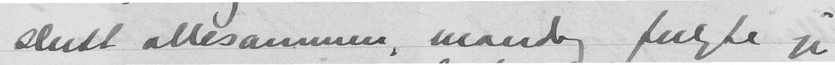slutt allesammen, mandag fulgte jeg
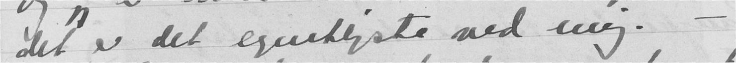det er det egentligste ved mig. -
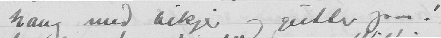hang med bikjer og gutter ogsaa!
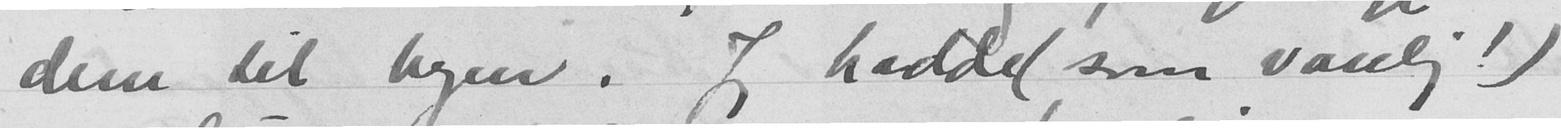dem til byen. Jeg hadde (som vanlig!)
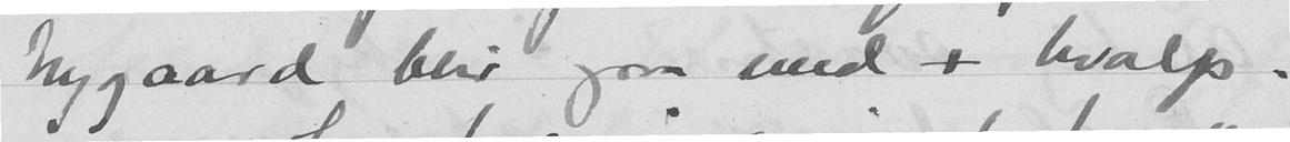Nygaard blir ogsaa med + hvalp.
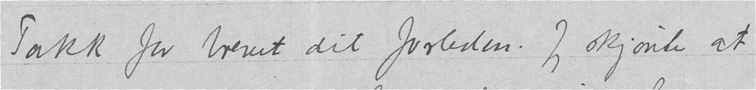Takk for brevet dit forleden. Jeg skjønte at
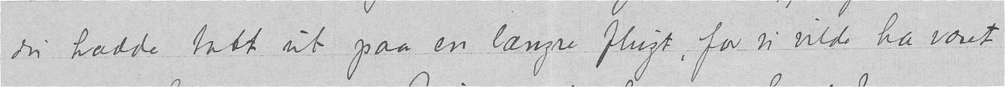du hadde tatt ut paa en længre flugt, for vi vilde ha været
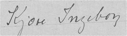Kjære Ingeborg
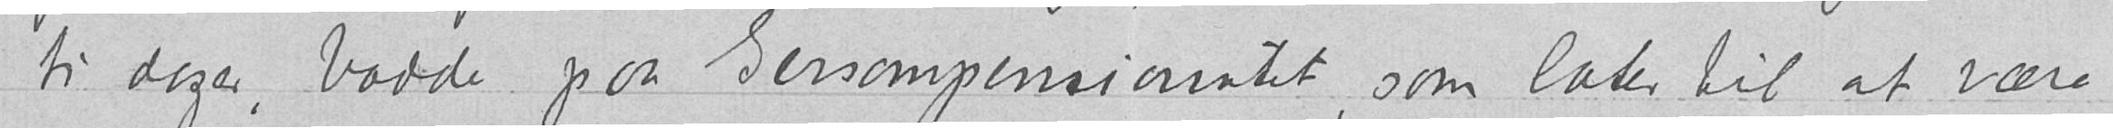ti dager, bodde paa Gersompensionatet, som later til at være
